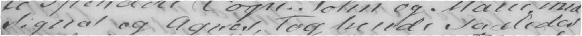Sigrid og Agnes, tog hende saaledes
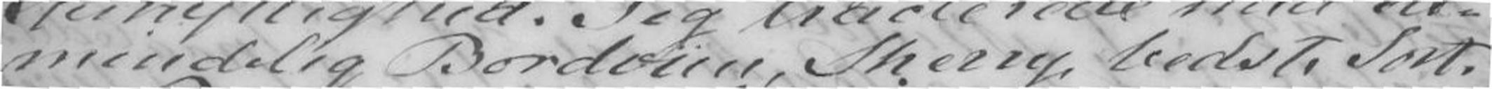mindelig Bordviin, Sherry bedste Sort,
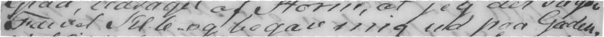Farvel Kl.6 og begav mig ud paa gaden,
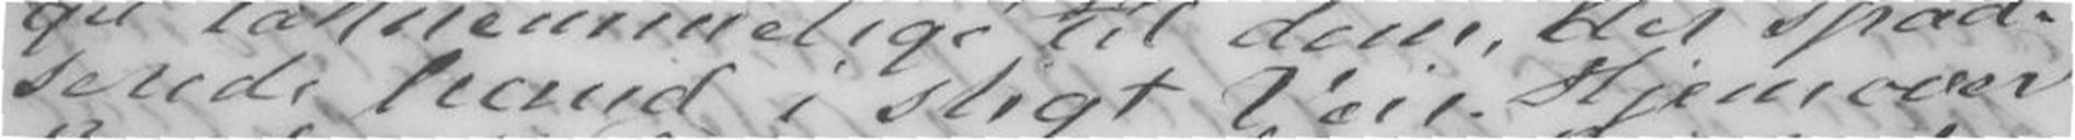serede hand i sligt Veir. Hjemover
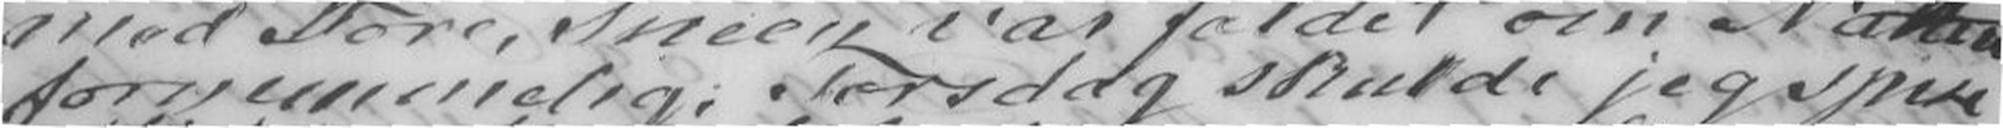fornnemelig, Torsdag skulde jeg spise
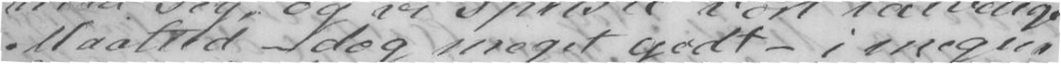Maaltid dog meget godt - i megen
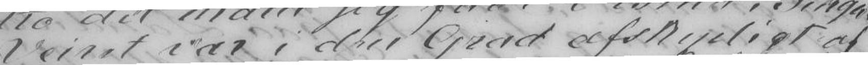Veiret var i den Grad afskyeligt af
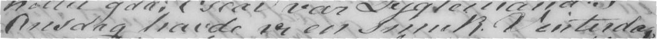Onsdag havde vi en Smuk Vinterdag
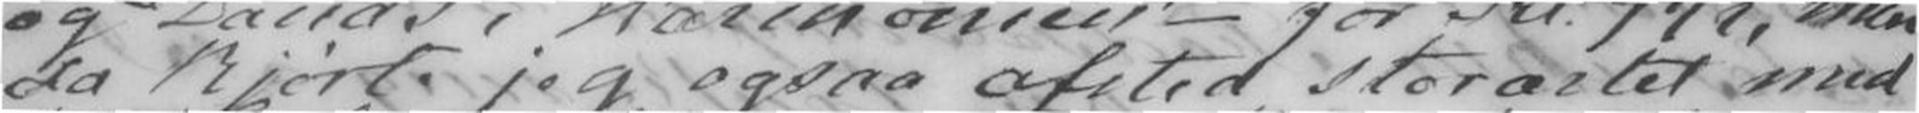da kjørtw jeg ogsaa afsted storartet med
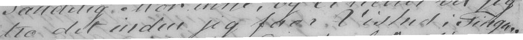tro det inden jeg faar Vished i Tingen.
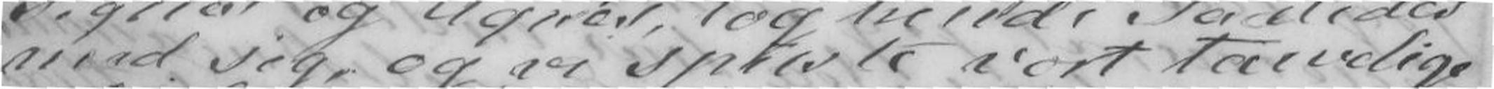med sig, og vi spiiste vort tarvelige
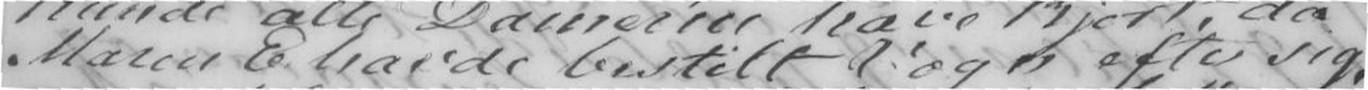Maren E. havde bestilt Vogn efter sig,
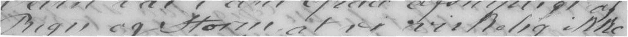Regn og Storm at vi virkelig ikke
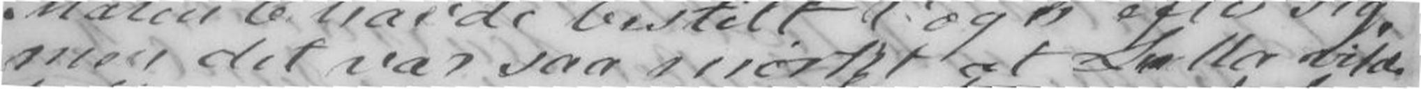men det var saa mørkt at Lulla vilde
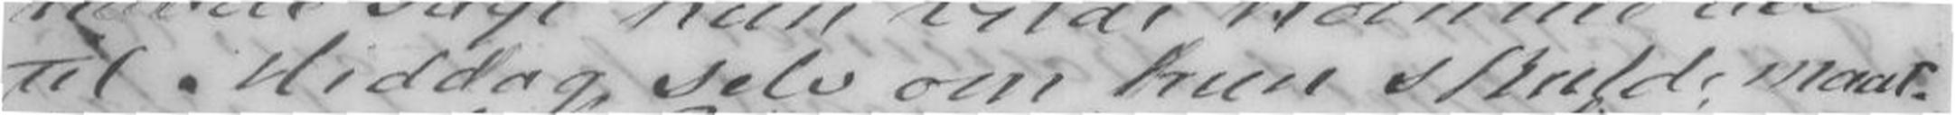til Middag selv om hun skulde maat-
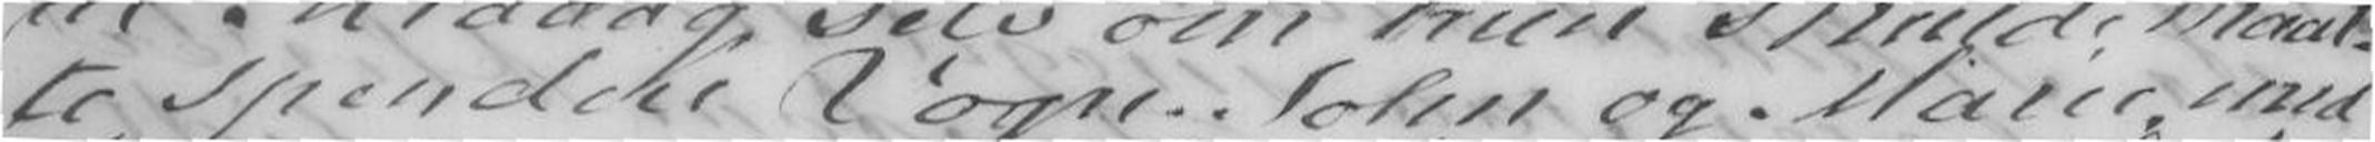te spendere Vogn. John og Marie med
